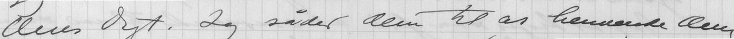Deres digt. Og råder Dem til at henvende Dem
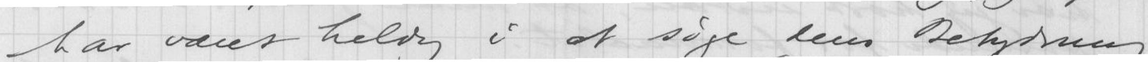har været heldig i at søge dens betydning
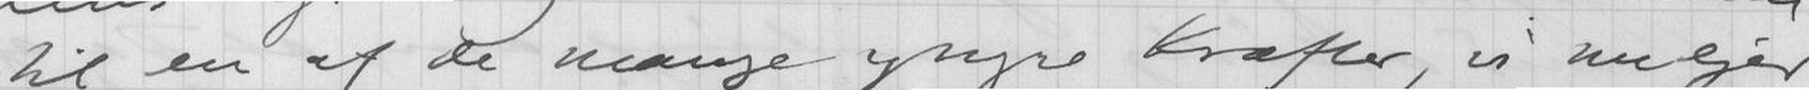til en af de mange yngre Krafter, vi
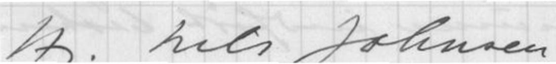Hr. Nils Johnsen
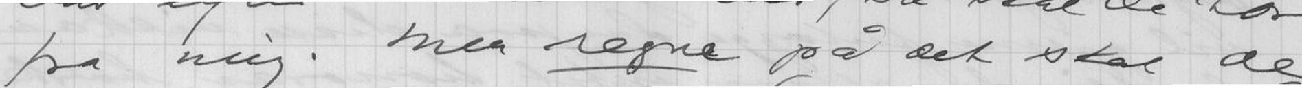på mig. Men tegne på det skal de
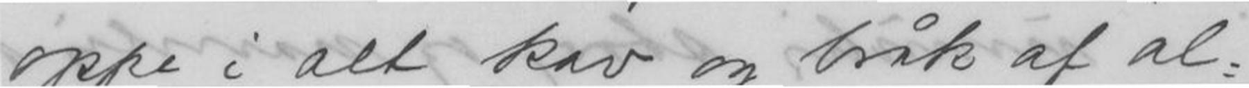oppe i alt kav og bråk af al-
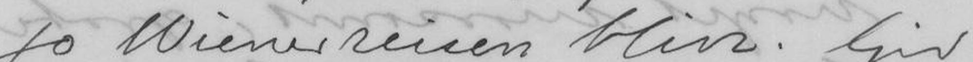jo Wienerreisen blive. Gid
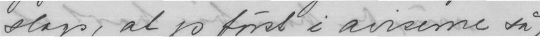slags, at jeg først i avisene så,
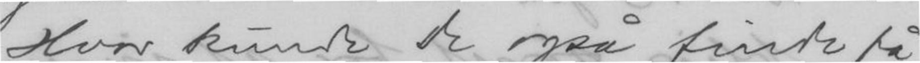Hvor kunde de også finde på
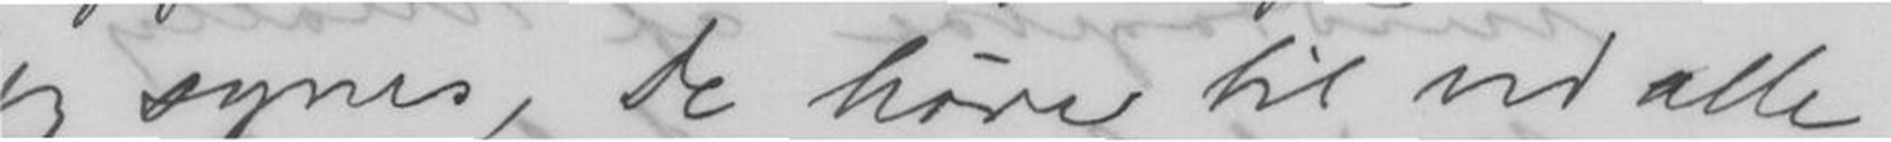jeg synes, de hører til ved alle
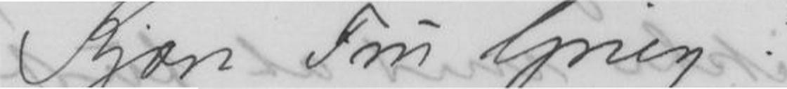Kjære Fru Grieg!
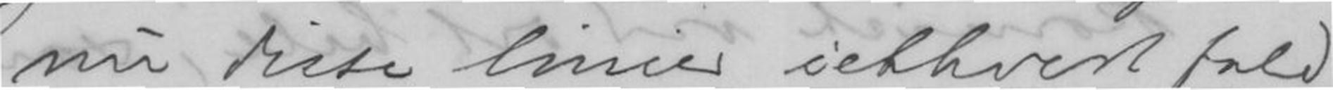nu disse linier iethvert fald
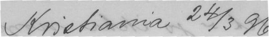Kristiania 24/3 96
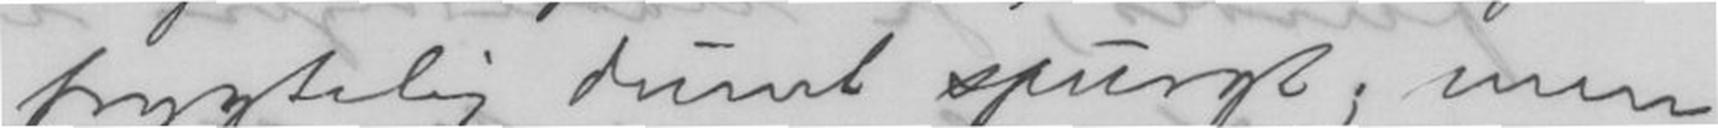frygtelig dumt spurgt; men
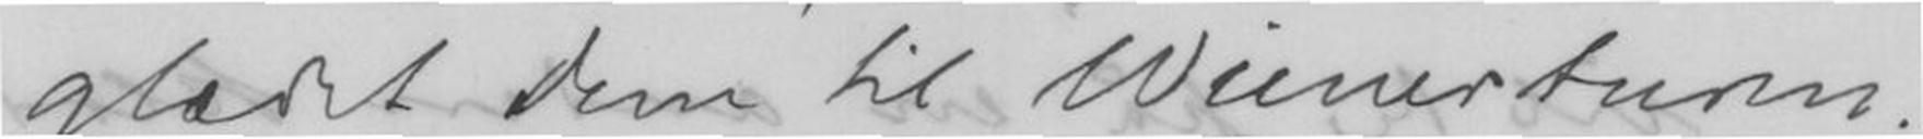glædet dem til Wienerturen.
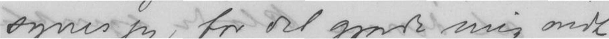synes jeg, for det gjorde mig ondt,
